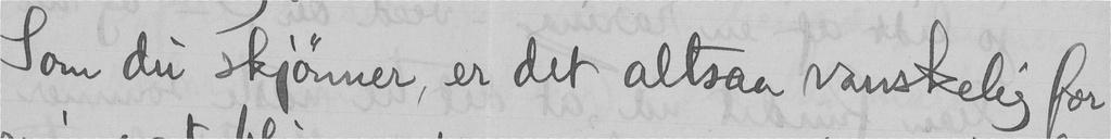Som du skjønner, er det altsaa vanskelig for
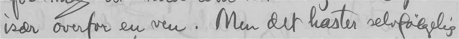især overfor en ven. Men det haster selvfølgelig
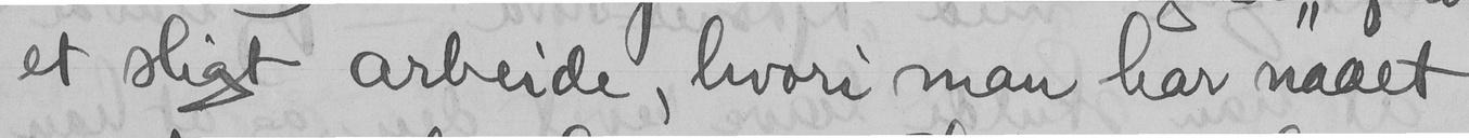et sligt arbeide, hvori man har naaet
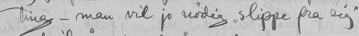ting - man vil jo nødig "slippe fra sig"
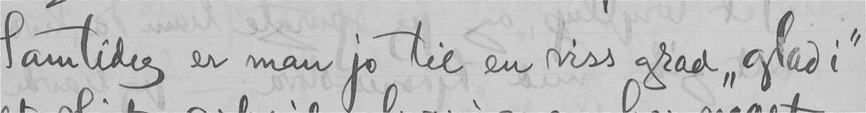Samtidig er man jo til en viss grad "glad i"
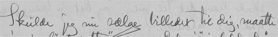Skulde jeg nu sælge billeder til dig, maatte
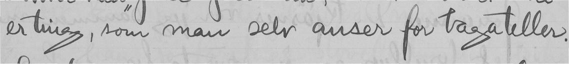er ting, som man selv anser for bagateller.
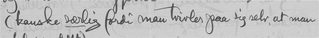(kanske særlig fordi man tvivler paa sig selv, at man
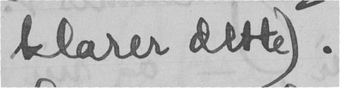klarer dette).
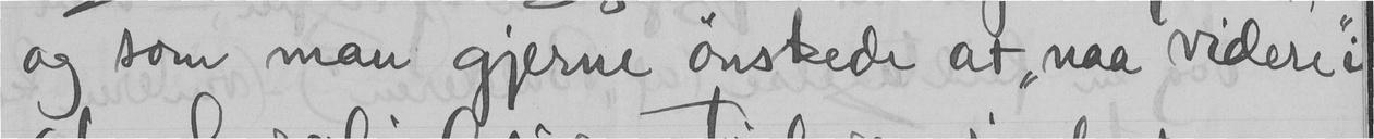og som man gjerne ønskede at "naa videre" i
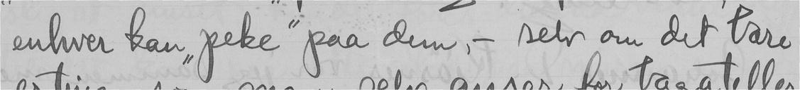enhver kan "peke" paa dem, - selv om det bare
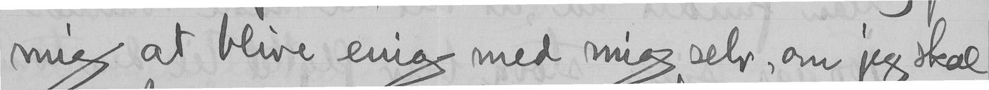mig at blive enig med mig selv, om jeg skal
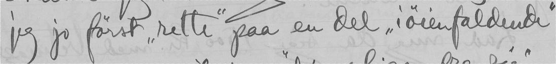jeg jo først "rette" paa en del "iøienfaldende"
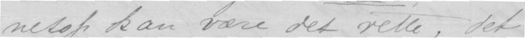netop kan være det rette, det
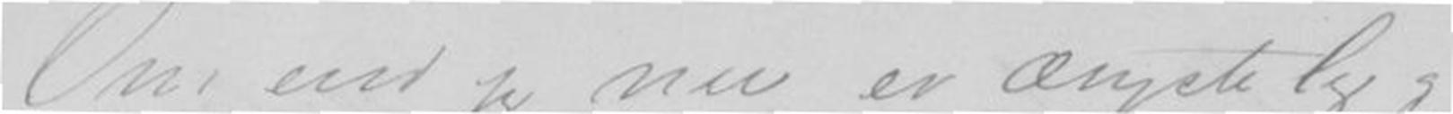Om end jeg nu er
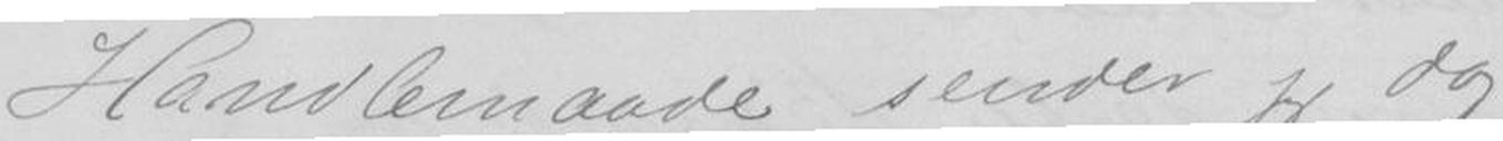Handlemaade, sender jeg dog
